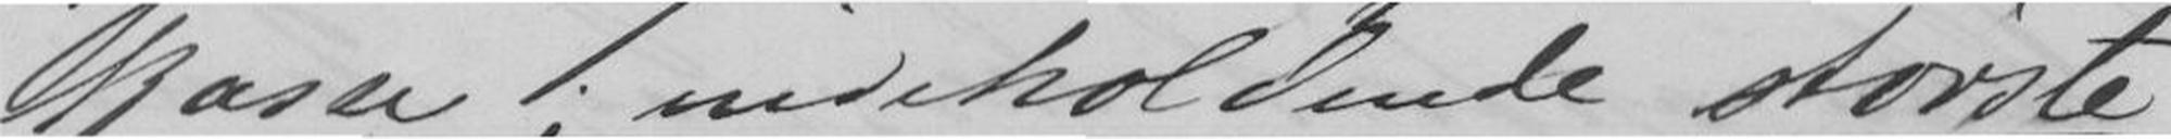kasse; indeholdende største-
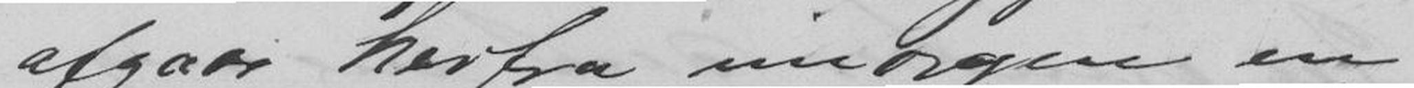afgaar herfra imorgen en
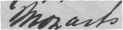Mozart
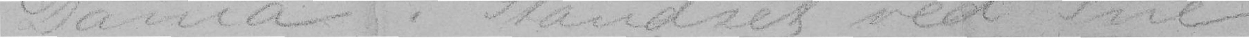Dania». Standset ved Sne.
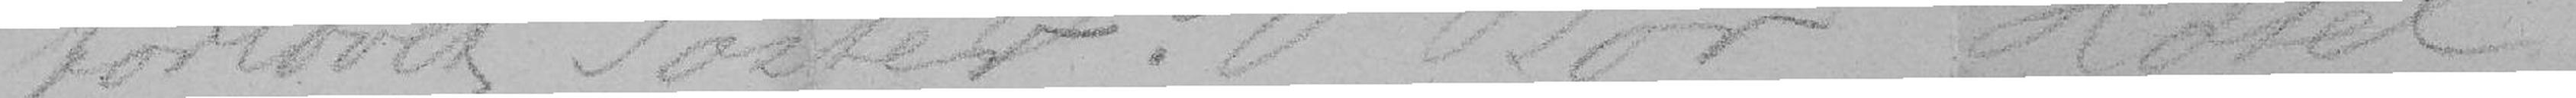forlovet Søster? Bor «Hotel
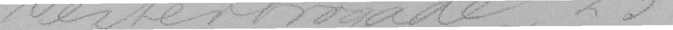Vesterbrogade 23
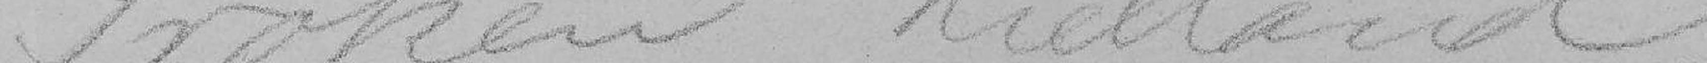Frøken Kielland
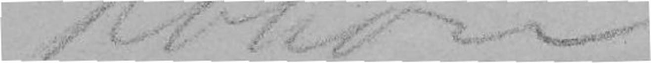Kbhavn
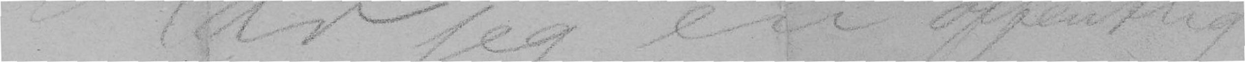Har jeg en offentlig
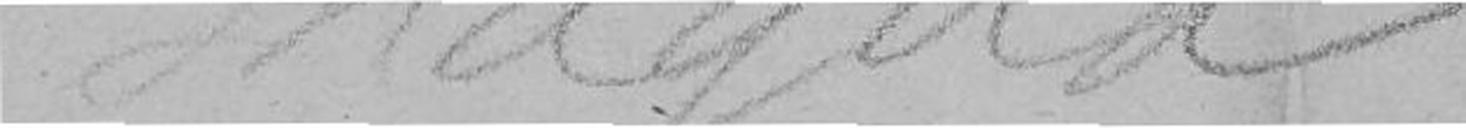Magda
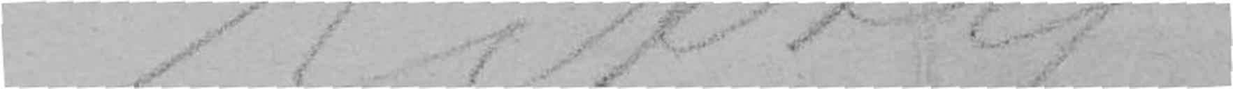Kitty
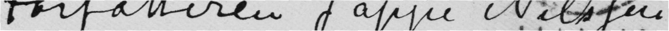Forfatteren Jappe Nilssen
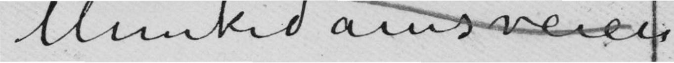Munkedamsveien
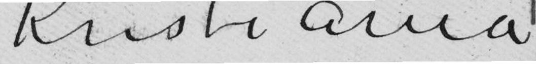Kristiania
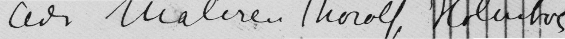Adr Maleren Thorolf Holmboe
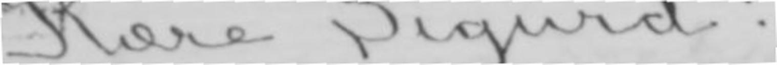Kære Sigurd!
